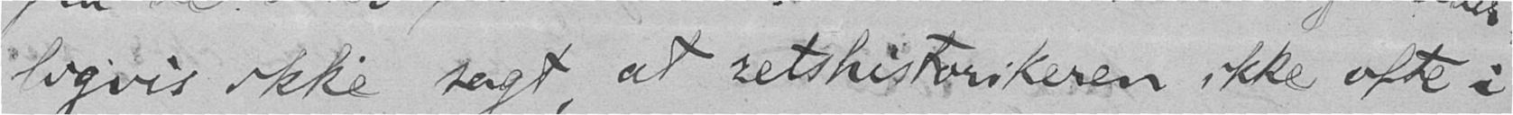ligvis ikke sagt, at retshistorikeren ikke ofte i
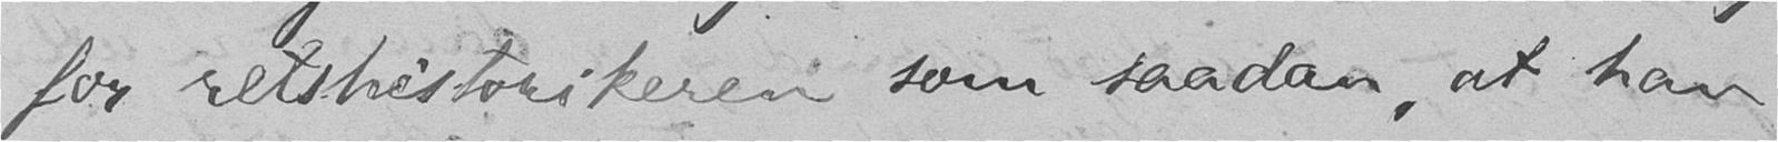for retshistorikeren som saadan, at han
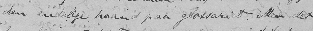den endelige haand paa glossariet. Men det
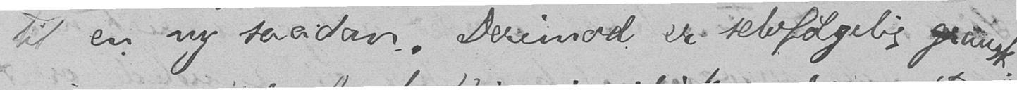til en ny saadan. Derimod er selvfølgelig gransk-
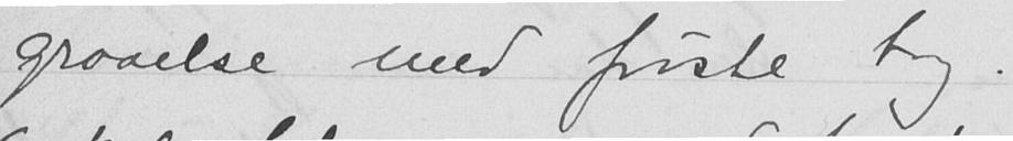gravelse med første tog.
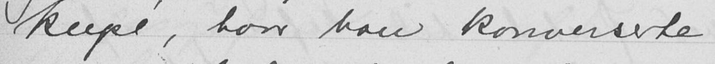kupé, hvor han konverserte
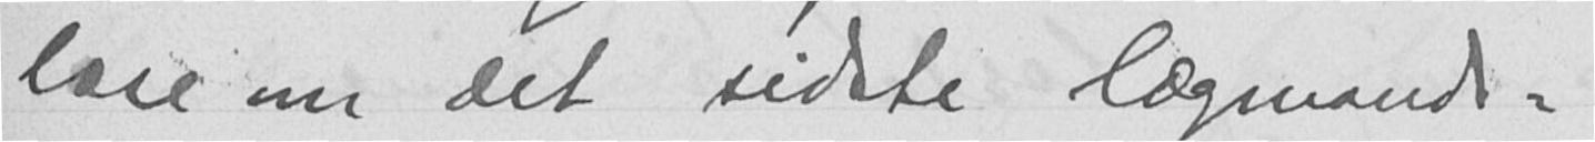læse om det sidste lægmands-
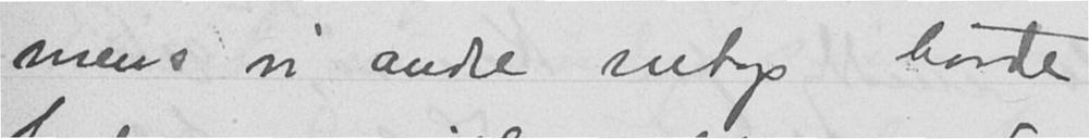mens vi andre netop havde
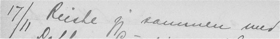17/11 Reiste jeg sammen med
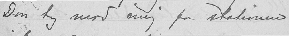Don tog mod mig paa stationen,
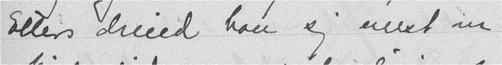Ellers dreied han sig mest om
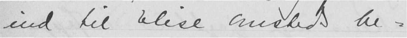ind til Elise Omsteds be-
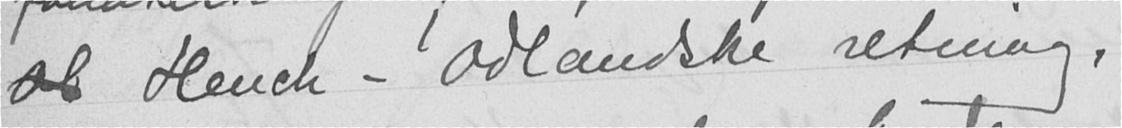Ol Heuch-Odlandske retning,
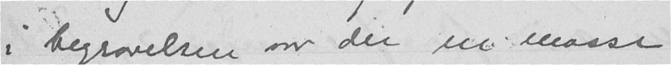i begravelsen var der en masse
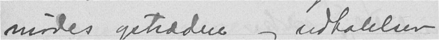mødes optræden og udtalelser
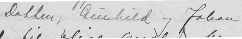Dotten, Gunhild og Johan
